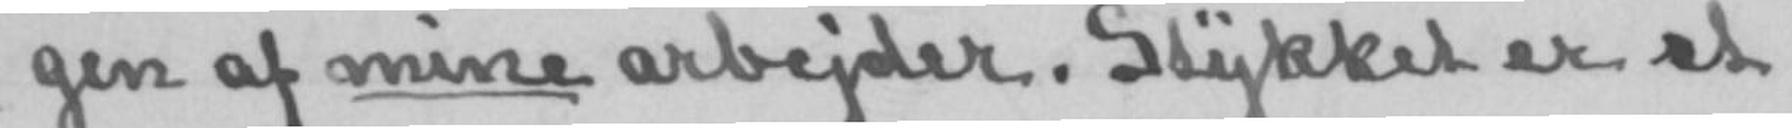gen af mine arbejder. Stykket er et
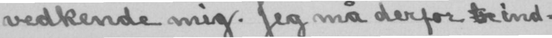vedkende mig. Jeg må derfor beind-
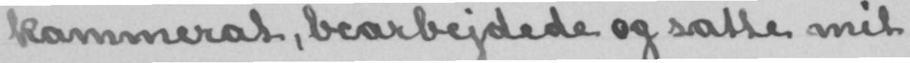kammerat, bearbejdede og satte mit
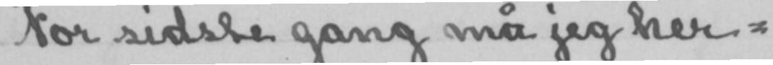For sidste gang må jeg her-
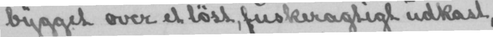bygget over et løst, fuskeragtigt udkast,
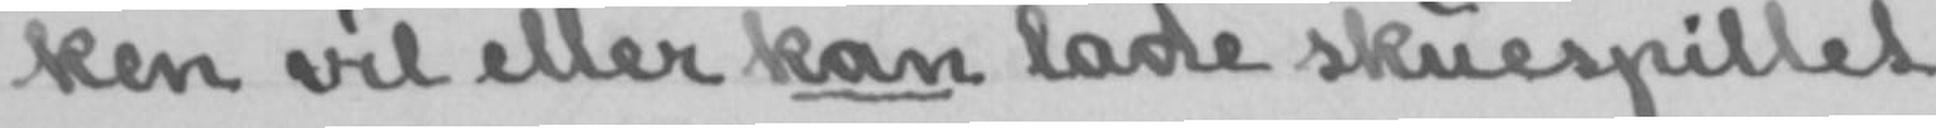ken vil eller kan lade skuespillet
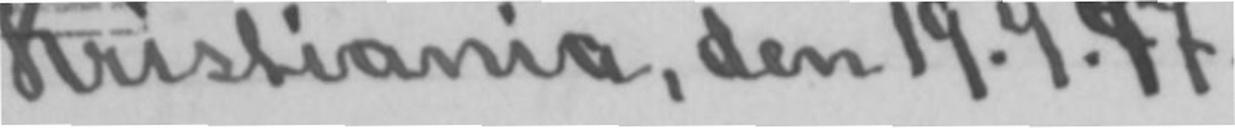Kristiania, den 19.9.97.
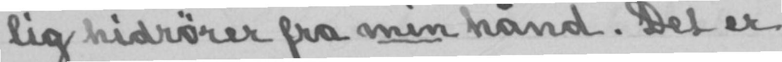lig hidrører fra min hånd. Det er
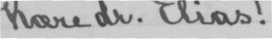Kære dr. Elias!
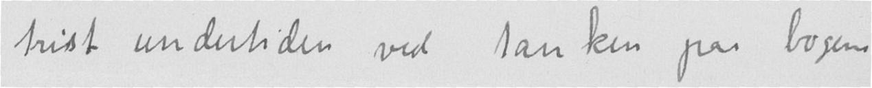trist undertiden ved tanken paa bogens
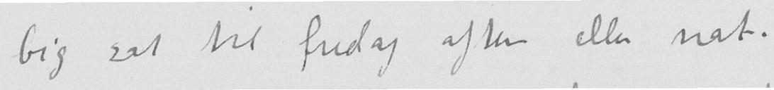big sat til fredag aften eller nat.
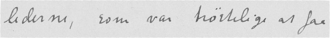lederne, som var trøstelige at faa.
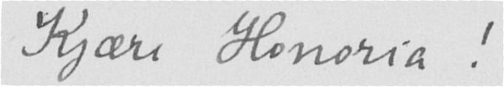Kjære Honoria!
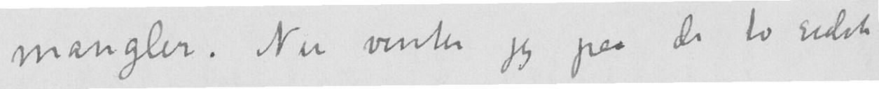mangler. Nu venter jeg paa de to sidste
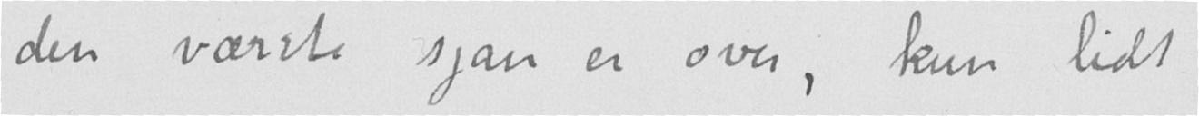den værste sjau er over, kun lidt
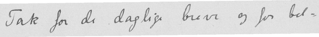Tak for de daglige breve og for bil-
